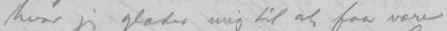hvor jeg glæder mig til at faa være
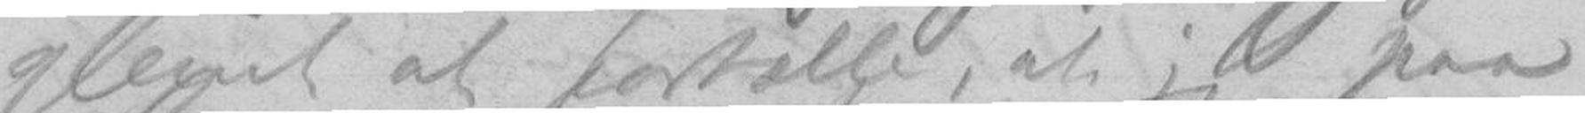glemt at fortælle, at jeg paa
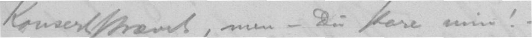Konsertstrævet, men - Du store min! -
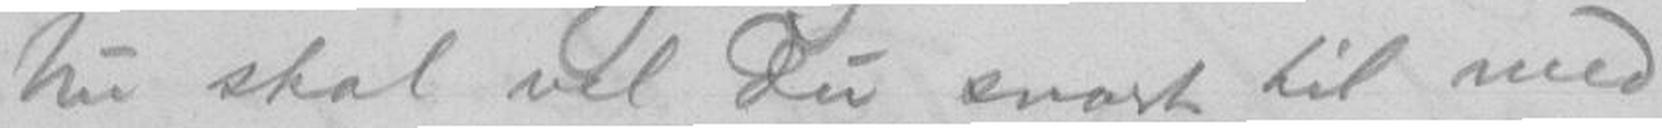Nu skal vel Du snart til med
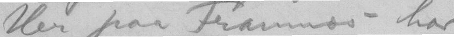Her paa Framnæs har
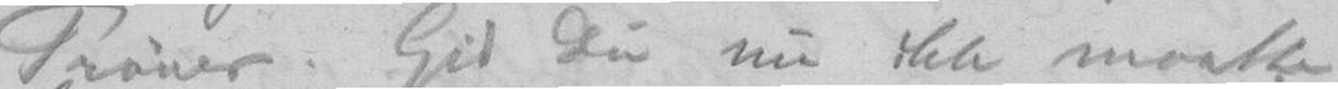Prøver. Gid Du nu ikke maatte
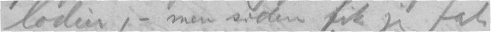lodier, - men siden fik jeg fat
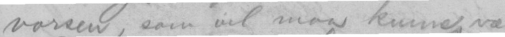vorsen, som vel maa kunne væ-
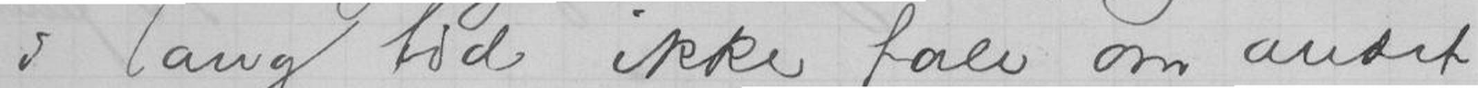i lang tid ikke tale om andet
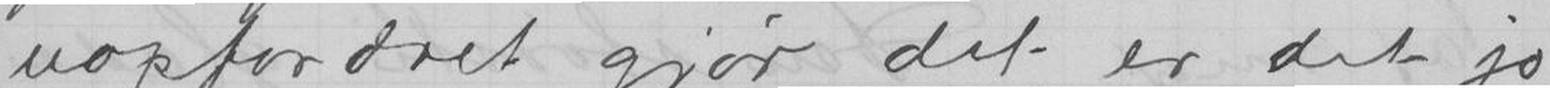uopfordret gjør det, er det jo
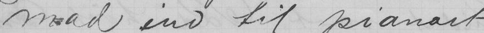mad ind til pianoet
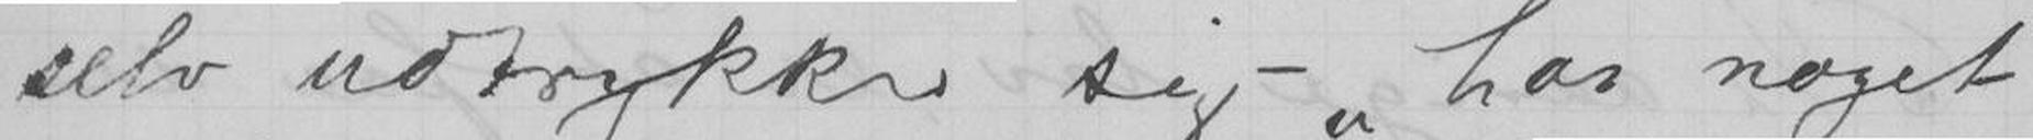selv udtrykker sig- har noget
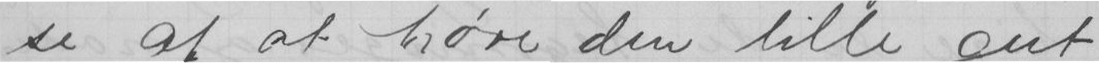se af at høre den lille gut
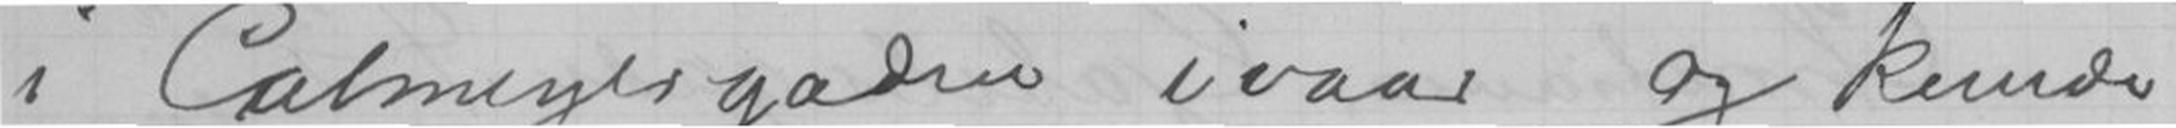i Calmeyergaden ivaar, og kunde
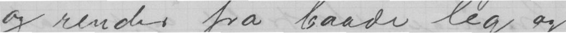og render fra baade leg og
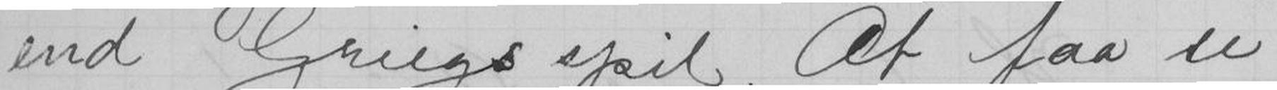end Griegs spil. At faa se
